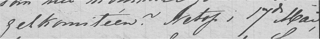getkomiteen? Netop i 17de Mai
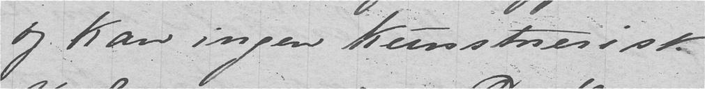og kan ingen kunstnerisk
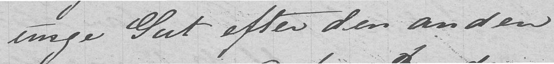unge Gut efter den anden
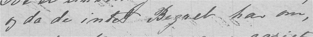og da de intet Begreb har om,
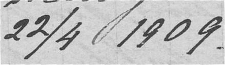22/4 1909.
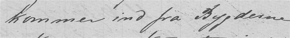kommer ind fra Bygderne
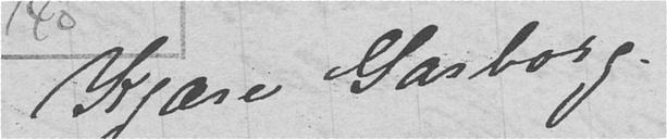Kjære Garborg!
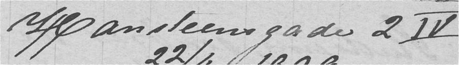Hansteensgade 2 IV
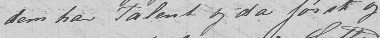dem har Talent og da først og
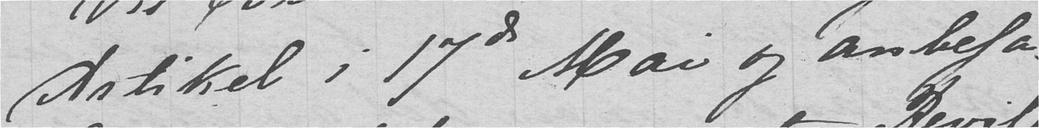Artikel i 17de Mai og anbefa-
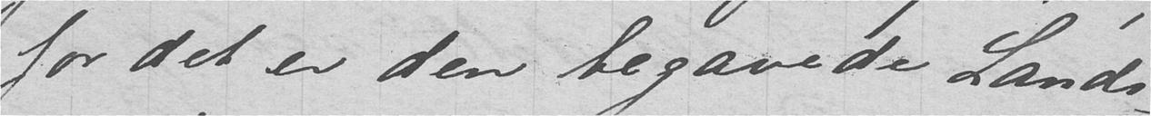for det er den begavede Lands-
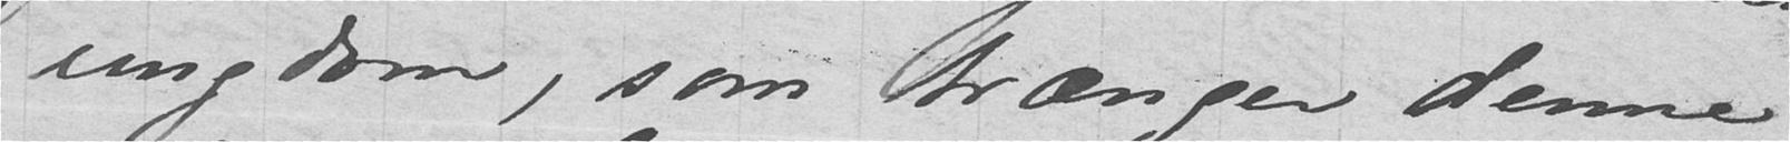ungdom, som trænger denne
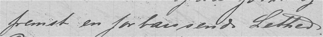fremst en forbausende Lethed.
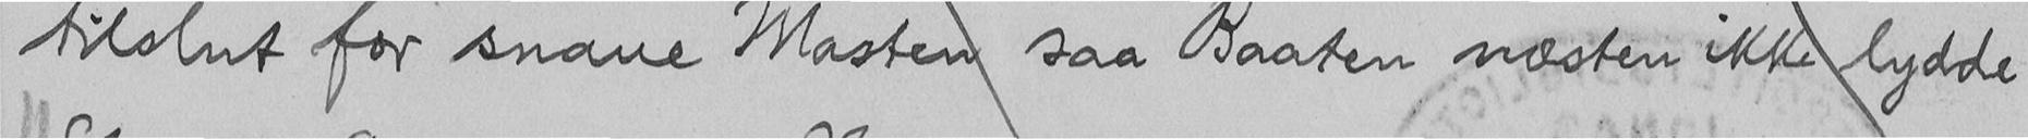tilslut for snaue Masten saa Baaten næsten ikke lydde
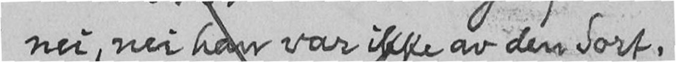nei, nei han var ikke av den Sort.
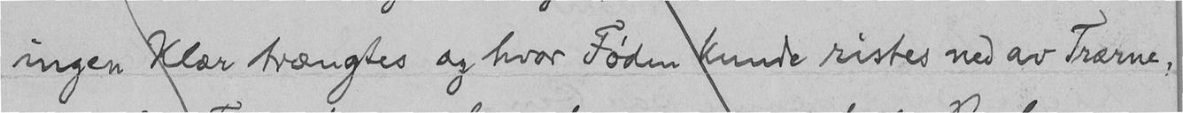ingen Klær trængtes og hvor Føden kunde ristes ned av Trærne,
